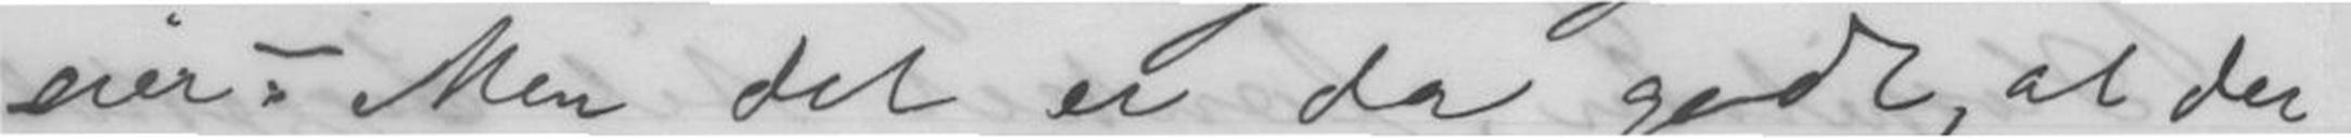eier.- Men det er da godt, at der
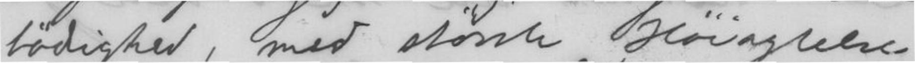bødighed, med største Høiagtelse
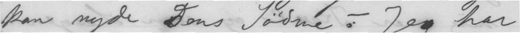kan nyde Dens Sødme. - Jeg har
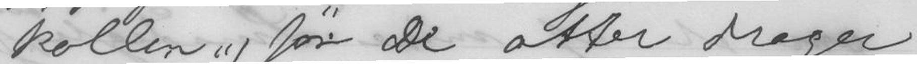kollen", før De atter drager
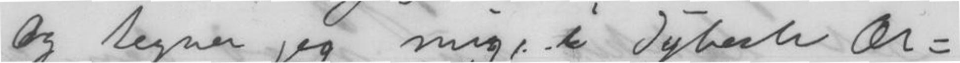Og tegner jeg mig, i dybesteÆr-
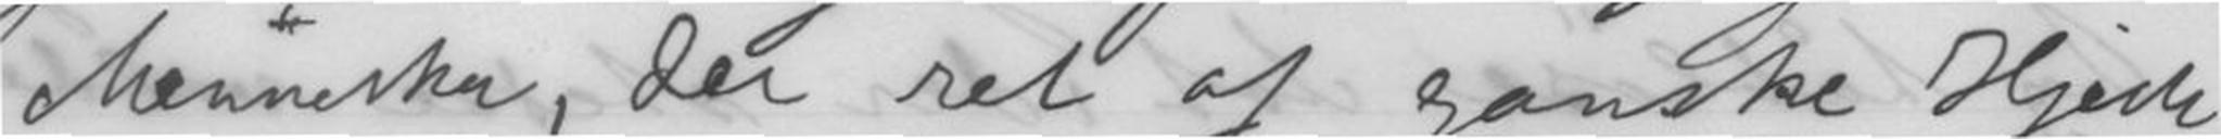Mennesker, der ret af ganske Hjerte
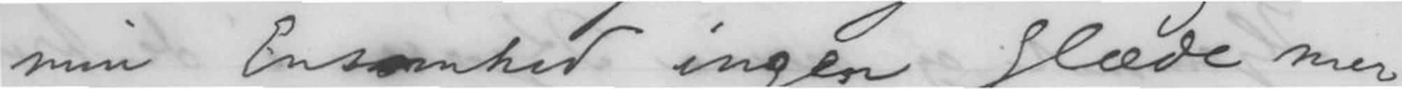min Ensomhed ingen Glæde mer
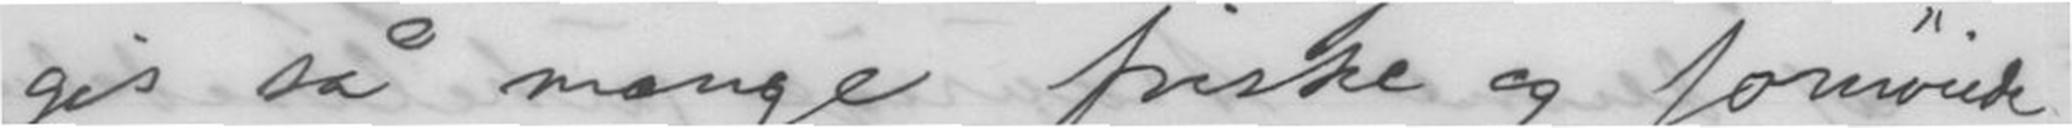gis så mange friske og fornøiede
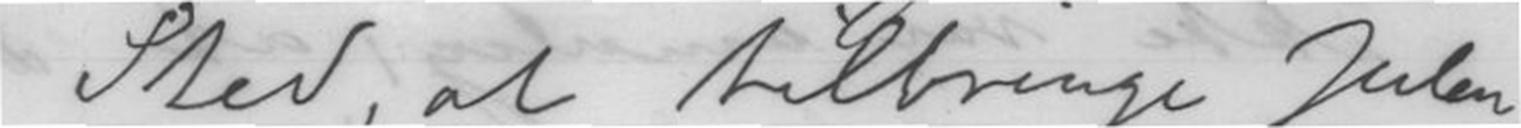pregtigere Sted, at tilbringe Julen
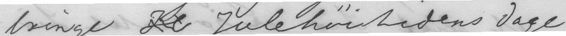bringe Julehøitidens Dage
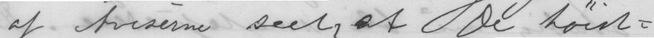af Aviserne seet, at De høist-
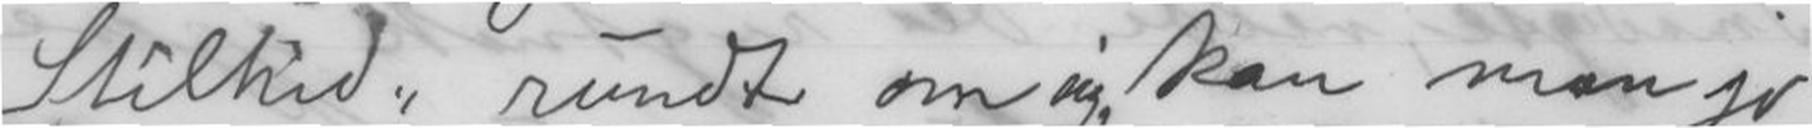Stillhed" rundt om sig, kan man jo
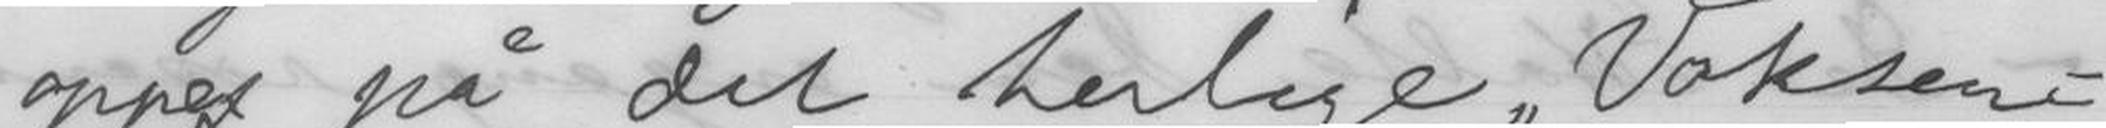oppe på det herlige "Voksen-
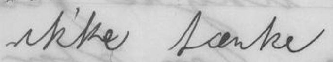ikke tænke sig! - Med mine
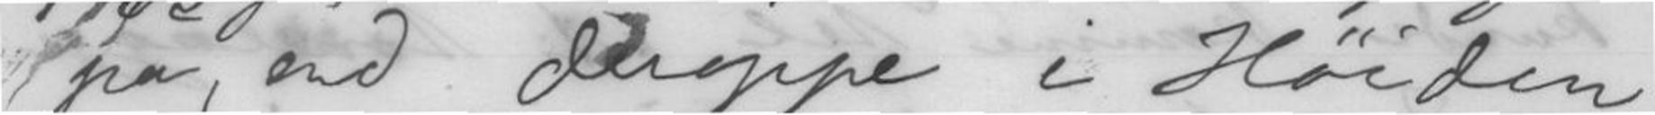på, end deroppe i Høiden
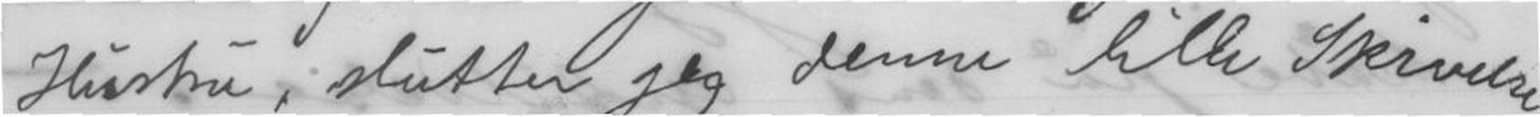Hustru; slutter jeg denne lille Skrivelse.
